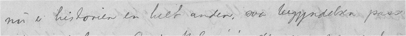nu er historien en helt anden, saa begyndelsen passer
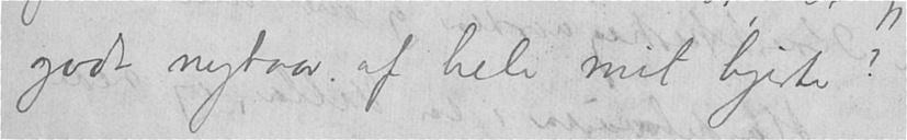godt nytaar af hele mit hjerte?
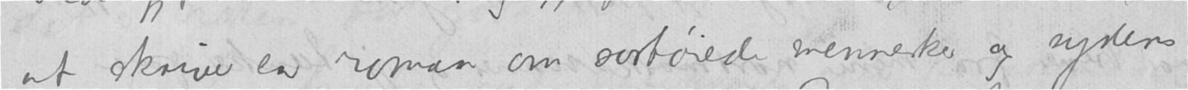at skrive en roman om sortøiede mennesker og sydens
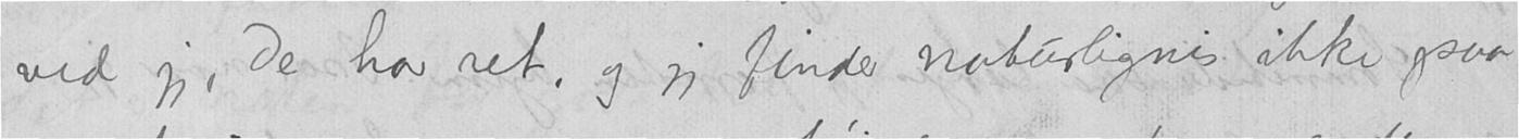ved jeg, De har ret, og jeg finder naturligvis ikke paa
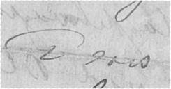Deres
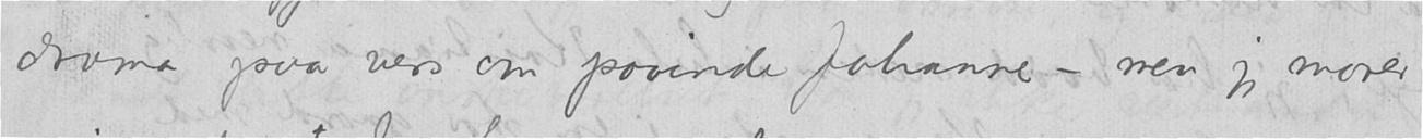drama paa vers om pavinde Johanne - men jeg morer
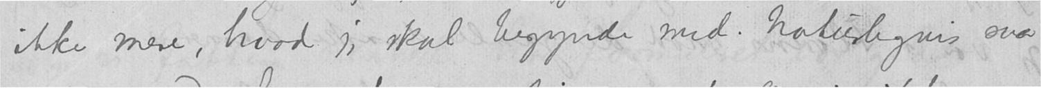ikke mere, hvad jeg skal begynde med. Naturligvis saa
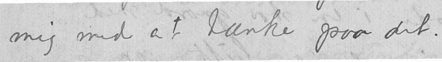mig med at tænke paa dit.
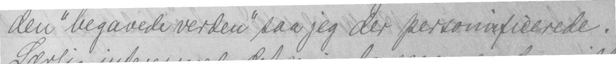den "begavede verden" saa jeg der personificerede.
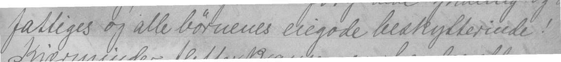fattiges og alle børnenes eiegode beskylterinde!
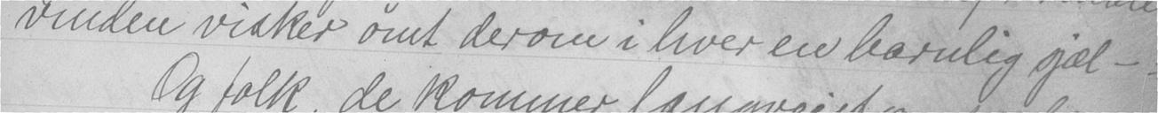vinden visker ømt derom i hver en barnlig sjæl -
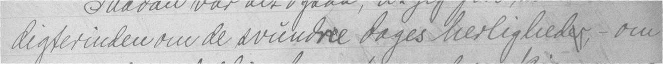digterinden om de svundne dages herligheder, - om
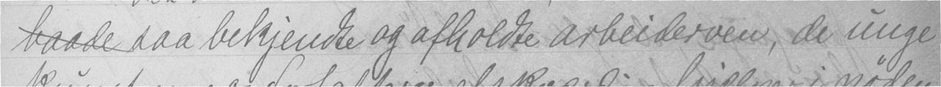baade saa bekjendte og afholdte arbeiderven, de unge
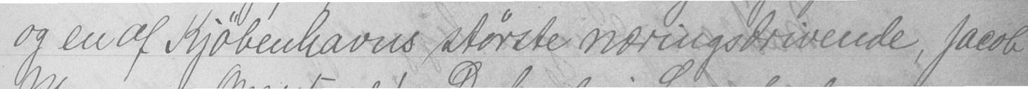og en af Kjøbenhavns største næringsdrivende, Jacob
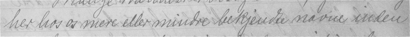her hos os mere eller mindre bekjendte navne inden
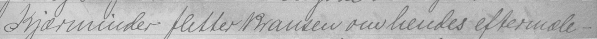Kjærminder fletter kransen om hendes eftermæle -
